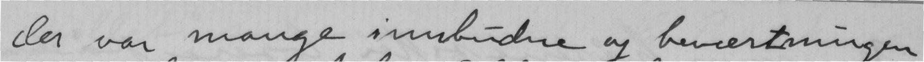der var mange innbudne og bevertningen
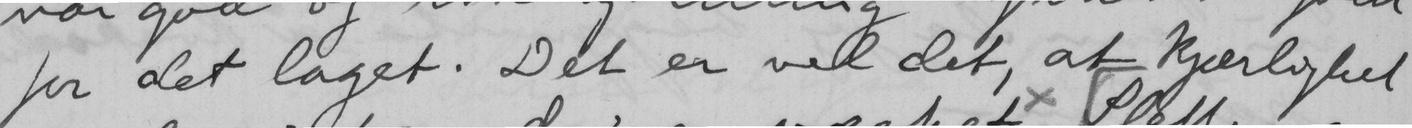for det laget. Det er vel det, at kjærlighet
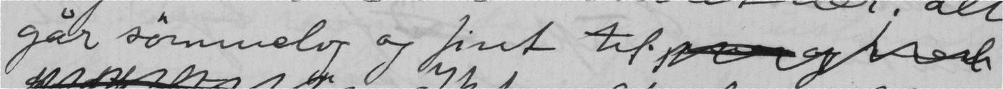går sømmelig og fint til
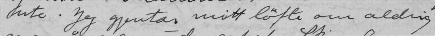ute. Jeg gjentar mitt løfte om aldrig
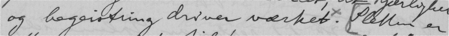og begeistring driver værket. Sletten er
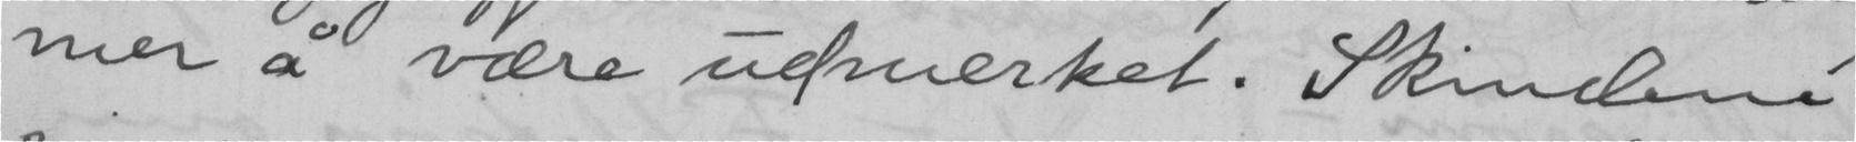mer å være udmerket. Skindene
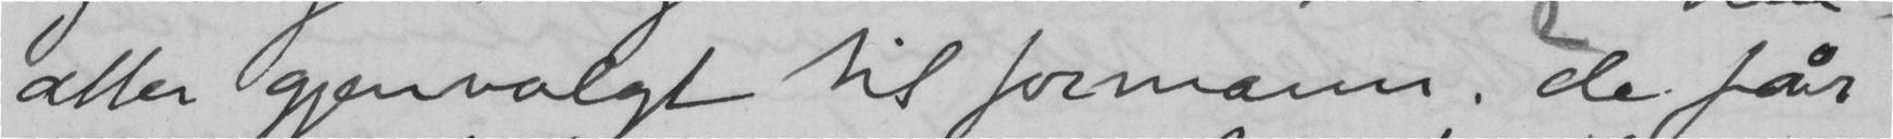atter gjenvalgt til formann; de får
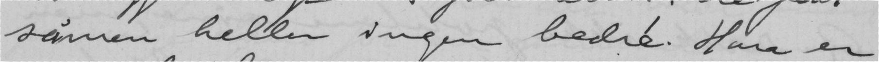såmen heller ingen bedre. Han er
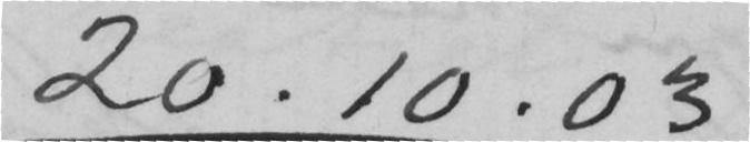20.10.03
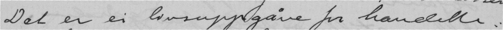Det er ei livsoppgåve for han dette:
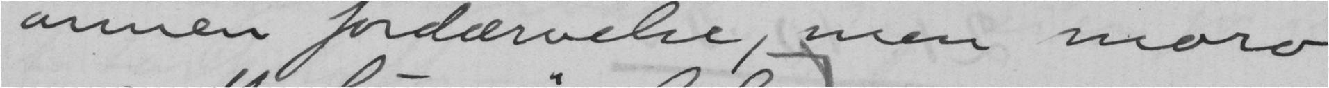annen fordærvelse, men moro
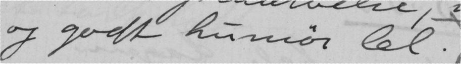og godt humør lel.
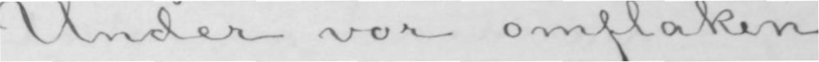Under vor omflaken
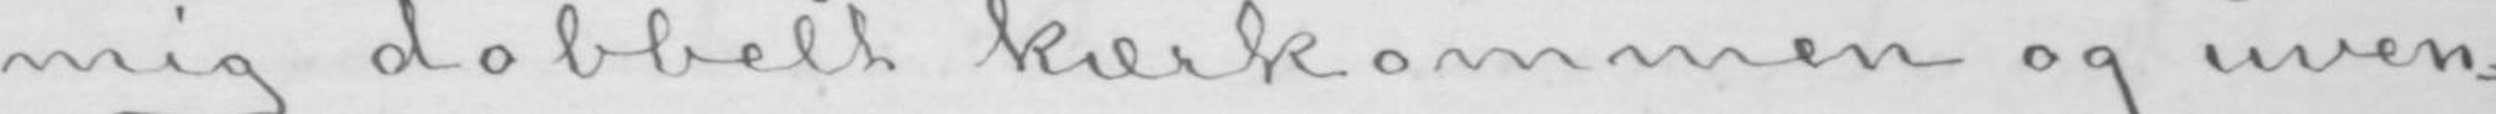mig dobbelt kærkommen og uven-
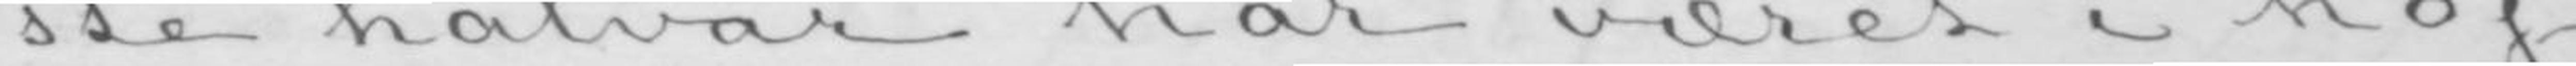ste halvår har været i høj
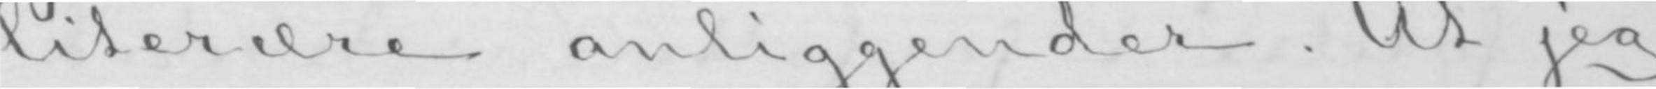literære anliggender. At jeg
